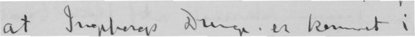at Ingeborgs Drenge er kommet i
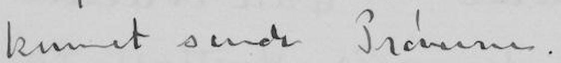kunnet sende Prøverne.
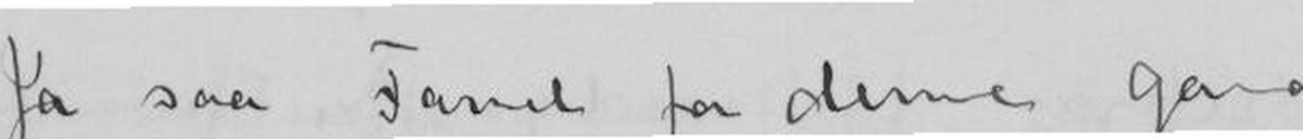Ja saa Farvel for denne gang
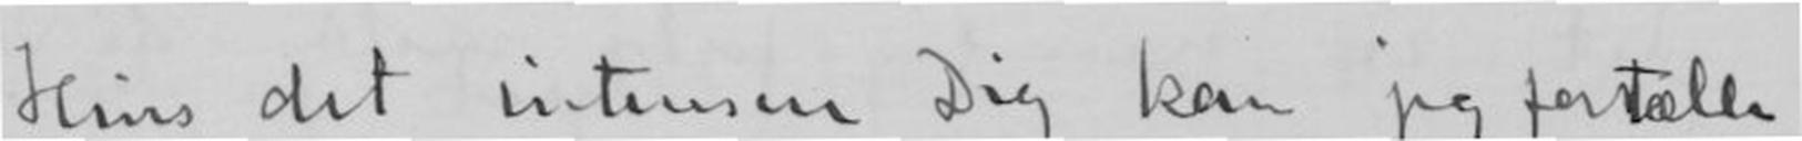Hvis det interesere Dig kan jeg fortælle
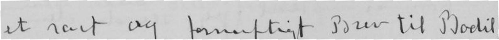et vart og fornuftigt Brev til Bodil,
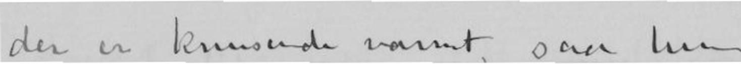der er knusende varmt, saa hun
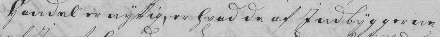Handel er nyttig, er hvad de af Indbyggerne
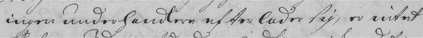ingen underhandlere efterlader sig, er intet
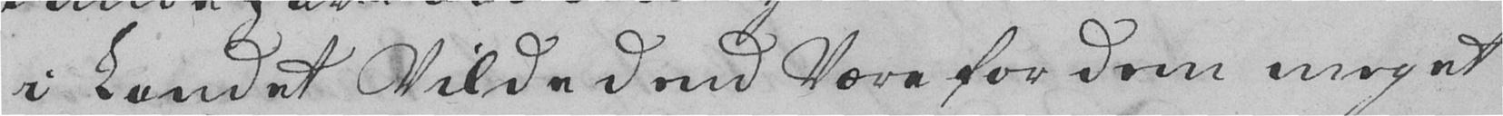i Landet vilde dend Være for dem meget
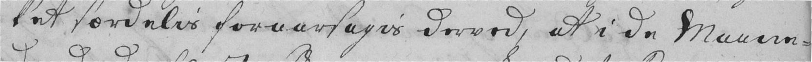ket særdelis foraarsages derved, at i de Maane-
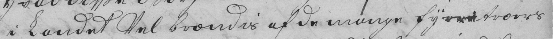i Landet vel brændis af de mange fyrretræers
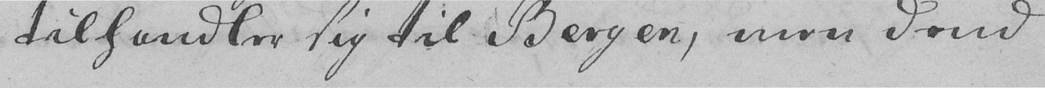tilhandler sig til Bergen, men dend
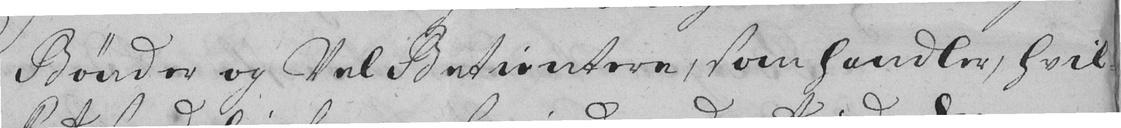Bønder og Vel Betientere, som handler, hvil-
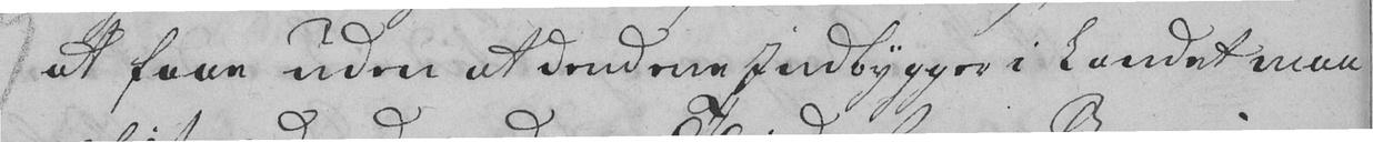at faae uden at dend ene Indbygger i Landet maa
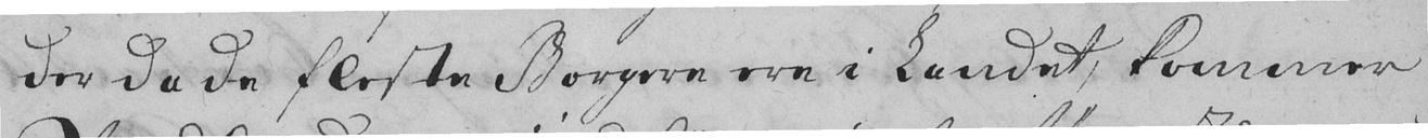der da de fleste Borgere ere i Landet, kommer
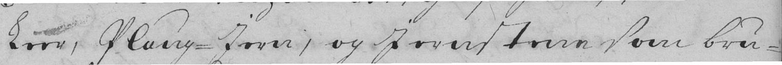Leer, Ploug-Iern, og Iernstene som bru-
